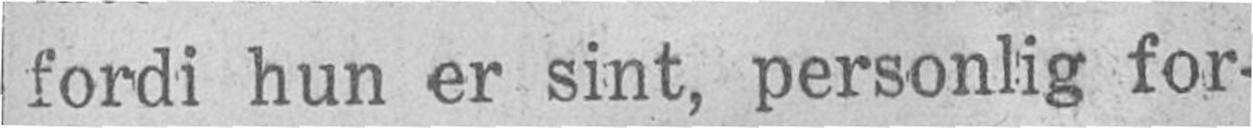fordi hun er sint, personlig for-
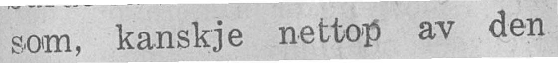som, kanskje nettop av den
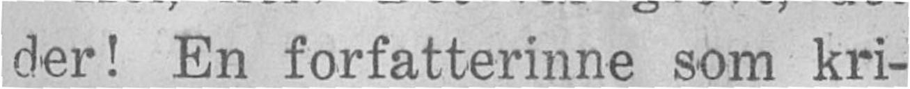der! En forfatterinne som kri-
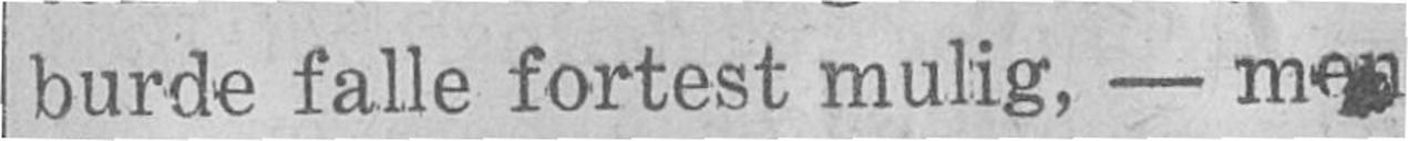burde falle fortest mulig, - men
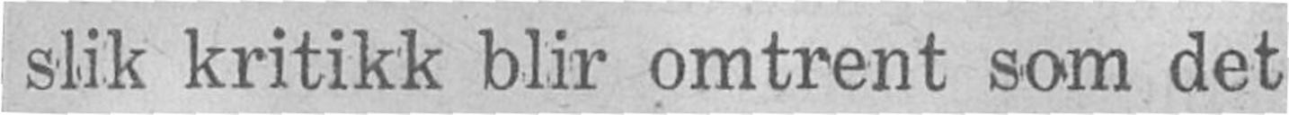slik kritikk blir omtrent som det
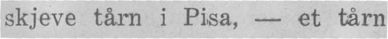skjeve tårn i Pisa, - et tårn
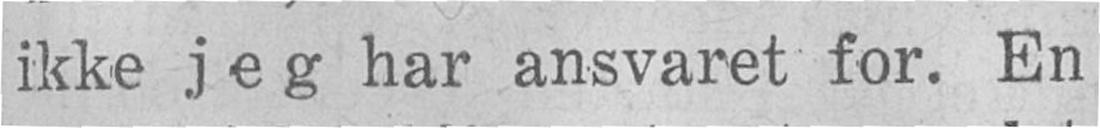ikke jeg har ansvaret for. En
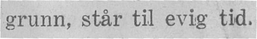grunn, står til evig tid.
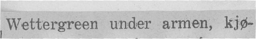Wettergreen under armen, kjø-
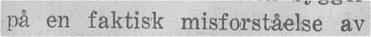på en faktisk misforståelse av
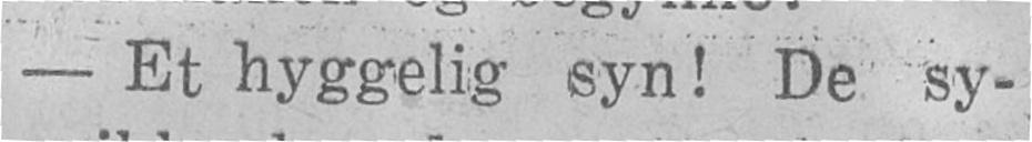- Et hyggelig syn! De sy-
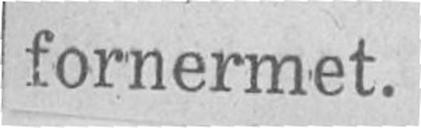fornermet.
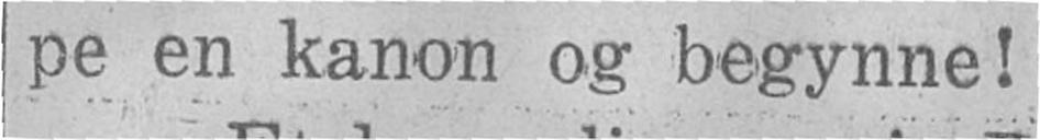pe en kanon og begynne!
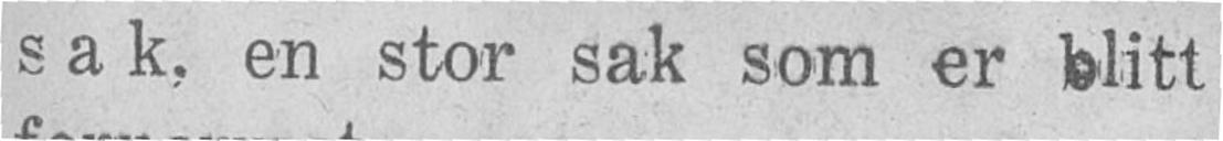sak, en stor sak som er blitt
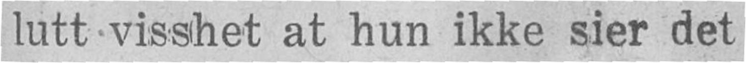lutt visshet at hun ikke sier det
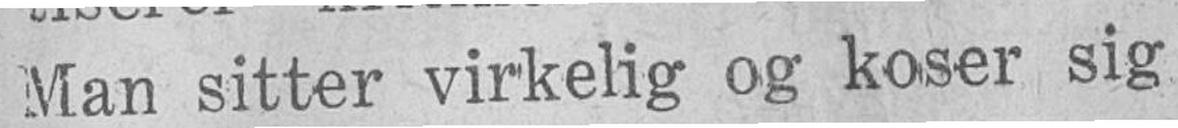Man sitter virkelig og koser sig
